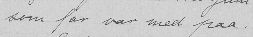som far var med paa.
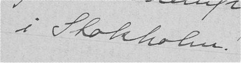i Stokholm!
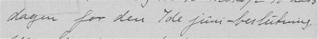dagen for den 7de juni-beslutning,
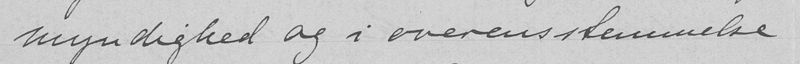myndighed og i overensstemmelse
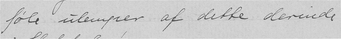føle ulemper af dette derinde
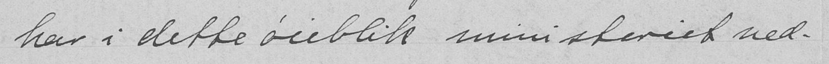har i dette øieblik ministeriet ned-
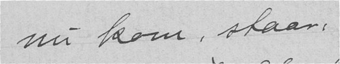nu kom, staar:
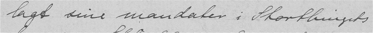lagt sine mandater i Storthingets
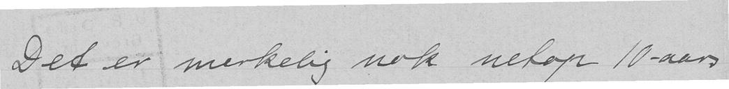Det er merkelig nok netop 10-aars
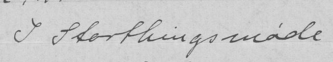I Storthingsmøde
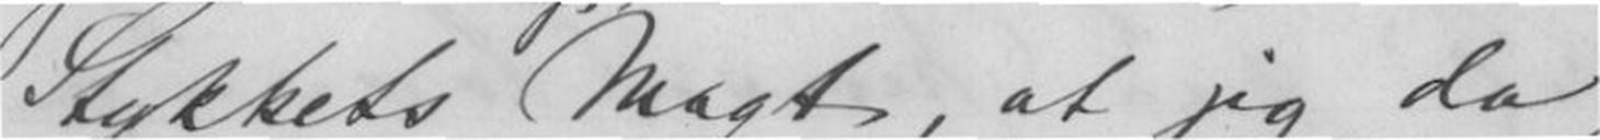Stykkets Magt, at jeg da
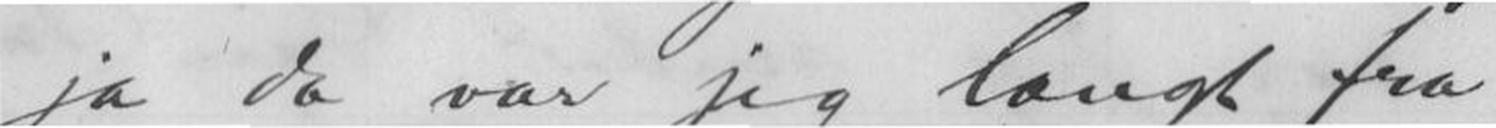ja da var jeg langt fra
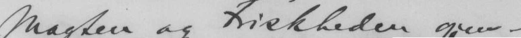Magten og Friskheden gjen-
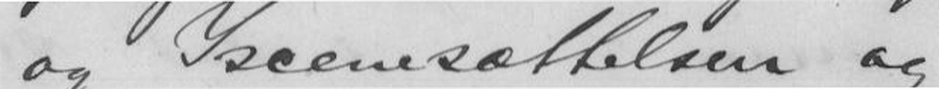og Iscenesættelsen og
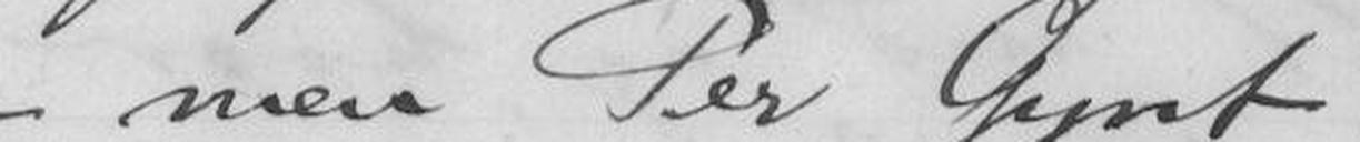- men Per Gynt
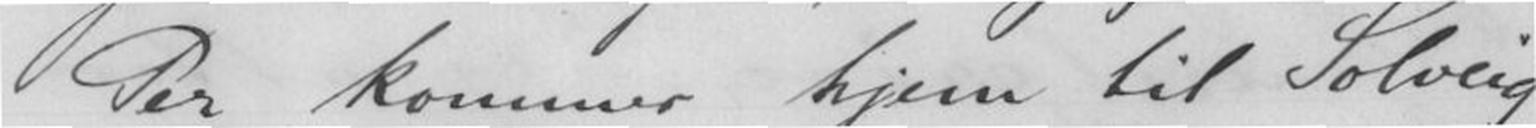Per kommer hjem til Solveig
